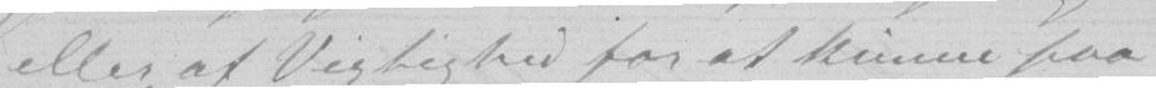eller af Vigtighed for at kunne faa
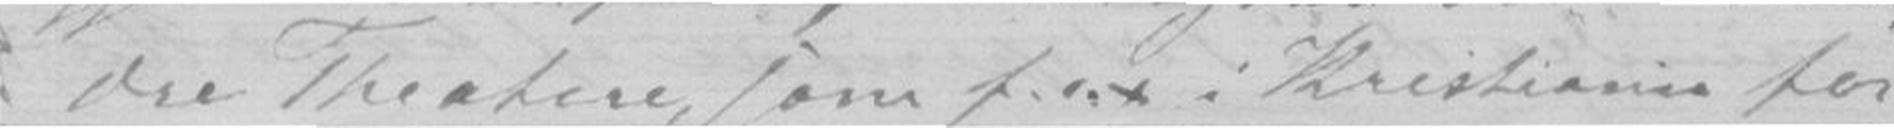dre Theatere, som f.e.x i Kristiania for
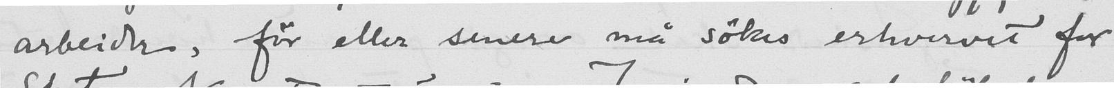arbeider, før eller senere må søkes erhvervet for
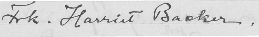Frk. Harriet Backer.
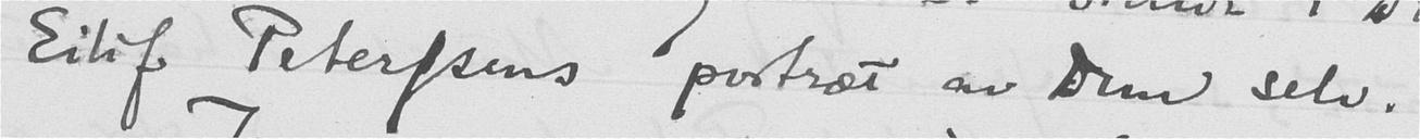Eilif Peterssens portræt av Dem selv.
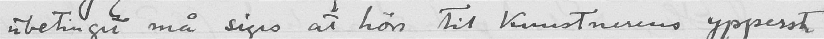ubetinget må siges at høre til kunstnerens ypperste
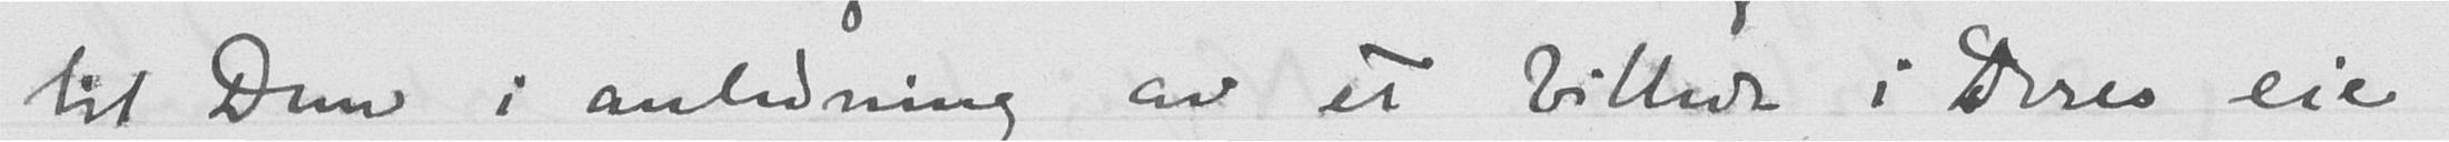til Dem i anledning av et billede i Deres eie -
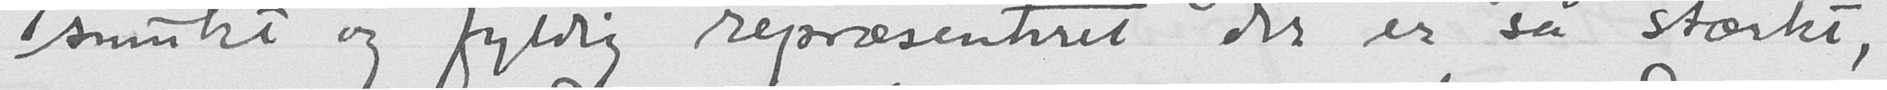smukt og fyldig repræsenteret der er så stærkt,
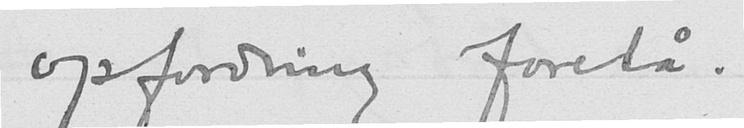opfordring forelå.
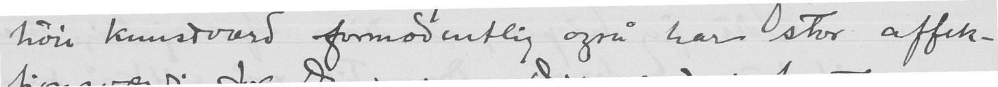høie kunstværd formodentlig også har stor affek-
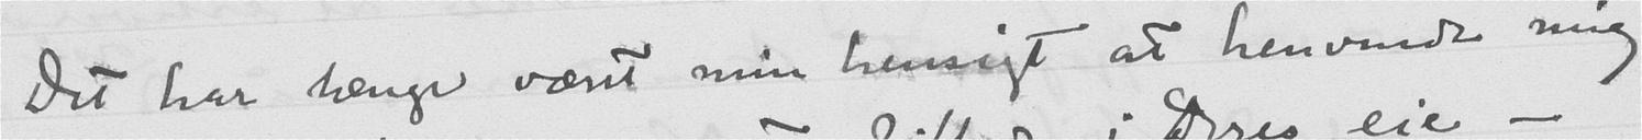Det har længe været min hensigt at henvende mig
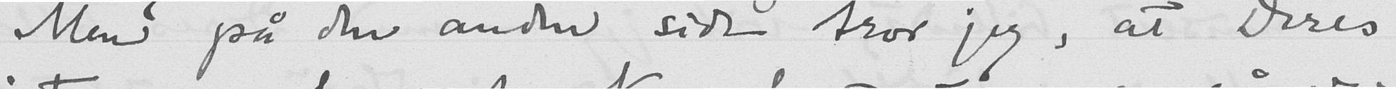Men på den anden side tror jeg, at Deres
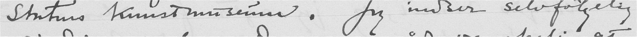statens kunstmuseum. Jeg indser selvfølgelig
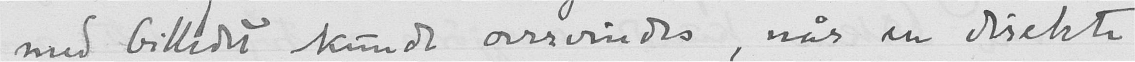med billedet kunde overvindes, når en direkte
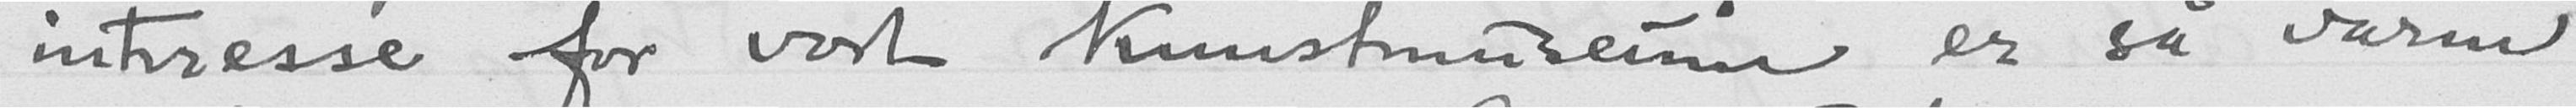interesse for vort kunstmuseum er så varm
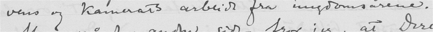vens og kamerats arbeide fra ungdomsårene.
